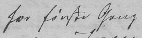for første Gang
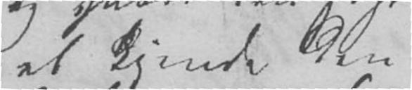at kjende den
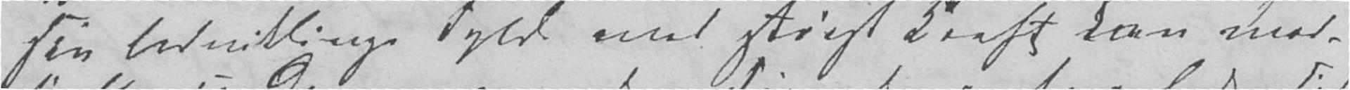sin Udviklings Fylde med størst Kraft kan mod-
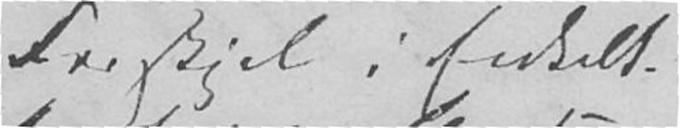Forskjel i Enkelt-
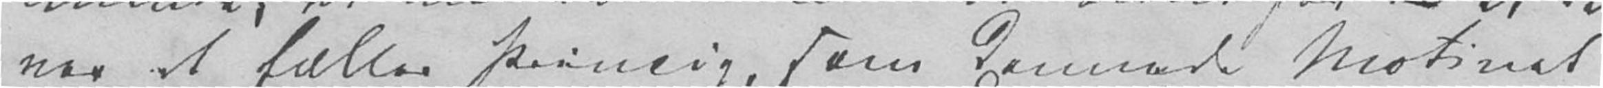var et fælles Princip, som dannede Motivet
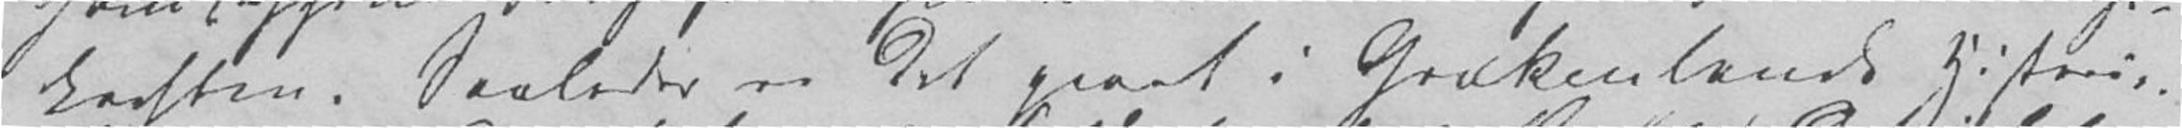kraften. Saaledes er det gaaet i Grækenlands Historie.
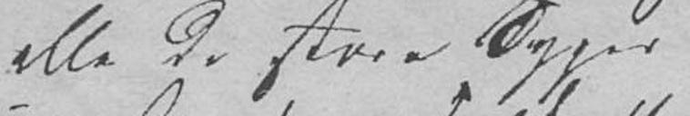alle de store Typer
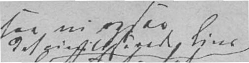det civiliserede Livs
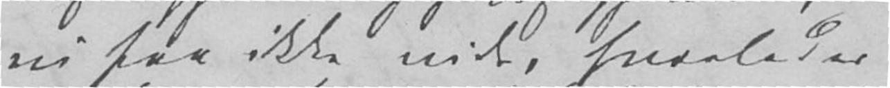vi faa ikke vide, hvorledes
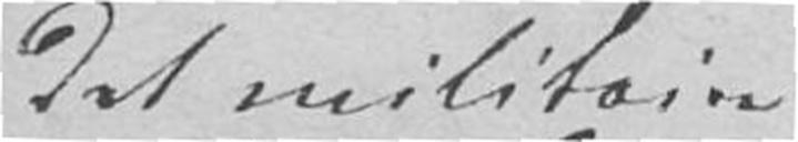det militaire
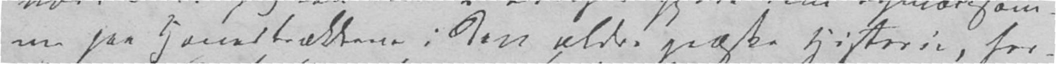me paa Hovedtrækkene i den ældre græske Historie, for-
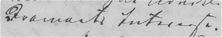Dramaets Interesse.
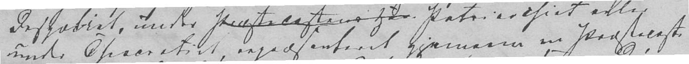under Theocratiet, repræsenteret gjemmen en Præstecaste.
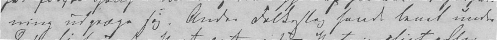ning udpræge sig. Andre Folkeslag havde levet under
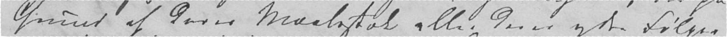Grund af deres Maalestok eller deres ydre Følger
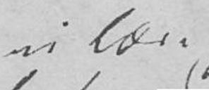vi lære
